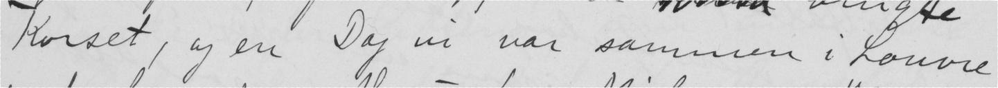Korset, og en Dag vi var sammen i Louvre
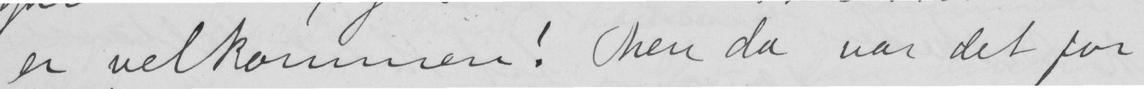er velkommen! Men da var det for
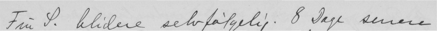Fru S. blidere selvfølgelig. 8 Dage senere
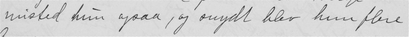misted hun ogsaa, og snydt blev hun flere
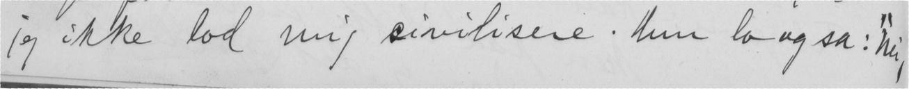jeg ikke lod mig civilisere. Hun lo og sa. "Nei,
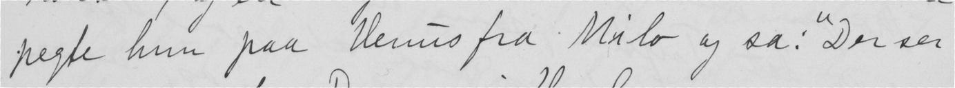pegte hun paa Venus fra Milo og sa: "Der ser
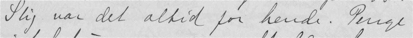Slig var det altid for hende. Penge
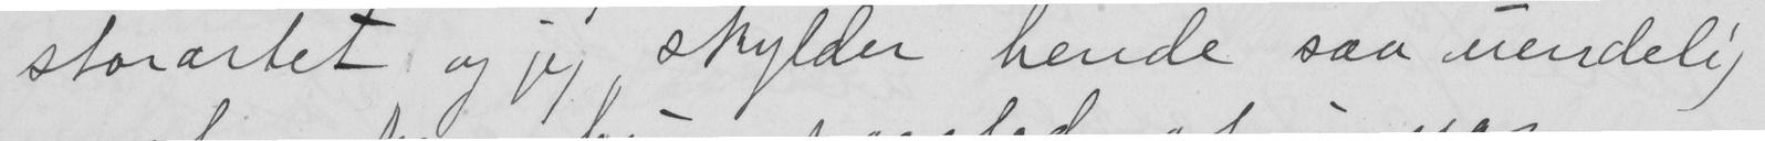storartet og jeg skylder hende saa uendelig
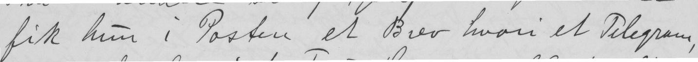fik hun i Posten et Brev hvor et Tilegram,
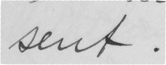sent.
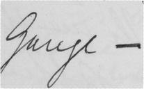Gange -
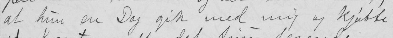at hun en Dag gik med mig og kjøbte
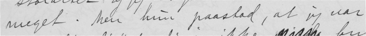meget. Men hun paastod, at jeg var
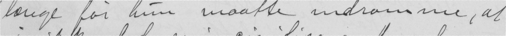længe før hun maatte indrømme, at
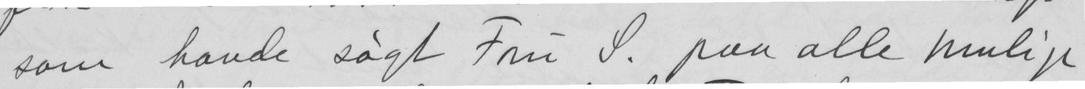som havde søgt Fru S. paa alle mulige
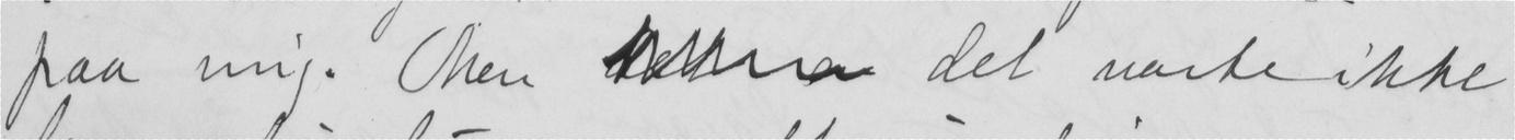paa mig. Men  det varte ikke
